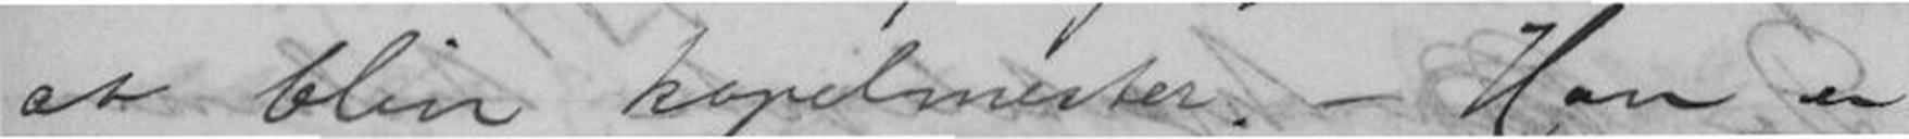at blive kapelmester. - Han er
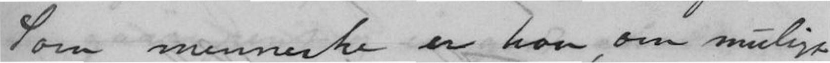- Som menneske er han, om muligt
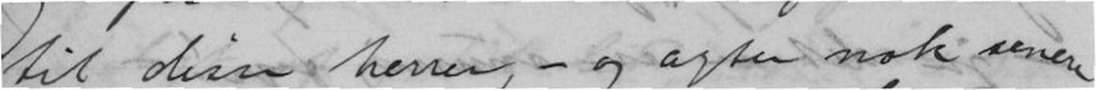til disse herrer, - og agter nok senere
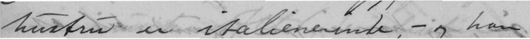hustru er italienerinde, - og han
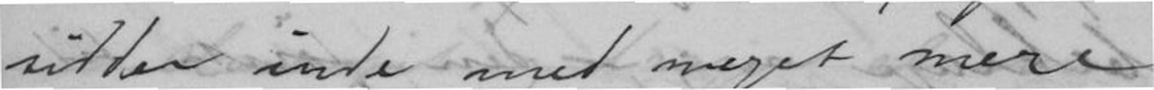sidder inde med meget mere
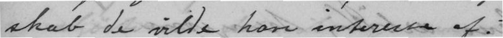skab de vilde have interesse af.
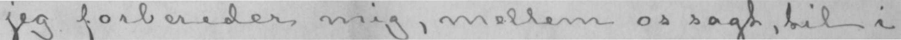jeg forbereder mig, mellem os sagt, til i
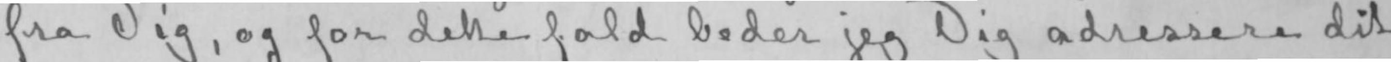fra Dig, og for dette fald beder jeg Dig adressere dit
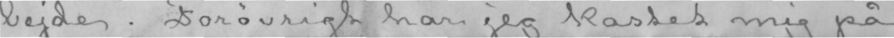bejde. Forøvrigt har jeg kastet mig på
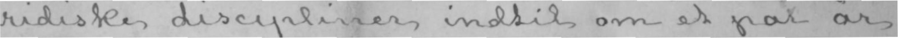ridiske discipliner indtil om et par år
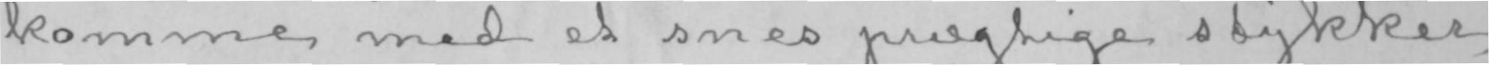komme med et snes prægtige stykker
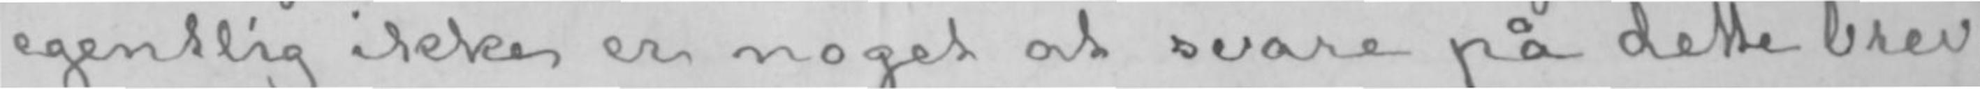egentlig ikke er noget at svare på dette brev,
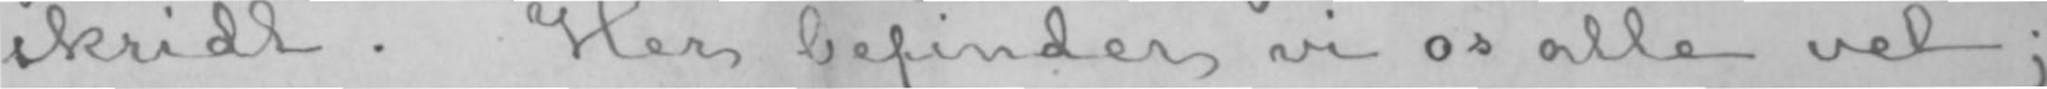skridt. Her befinder vi os alle vel;
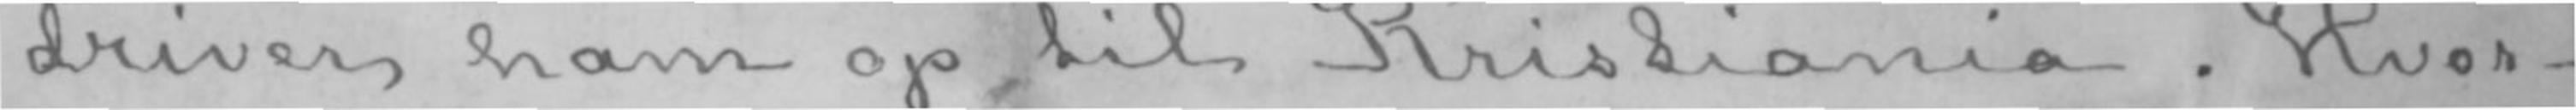driver ham op til Kristiania. Hvor-
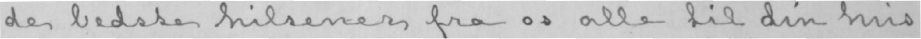de bedste hilsener fra os alle til din hus-
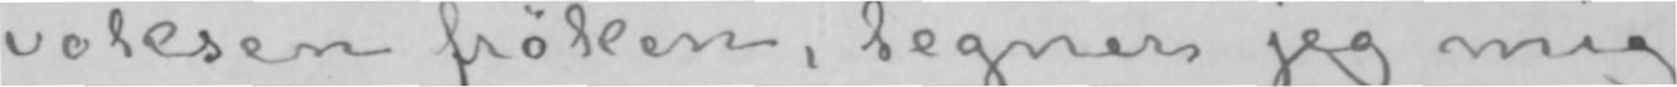voksen frøken, tegner jeg mig
